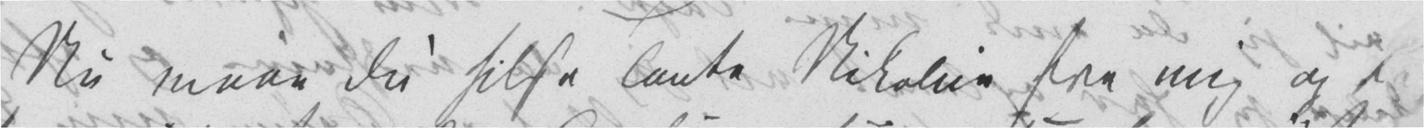Nu maae Du hilse Tante Nikoline fra mig og ta
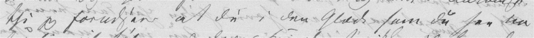thi jeg forudseer at Du i den Glæde som Du saa na-
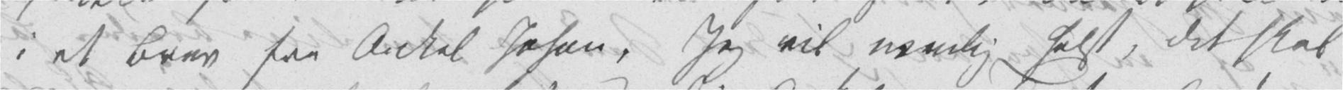i et Brev fra Onkel Johan, Jeg vil nemlig helst, det skal
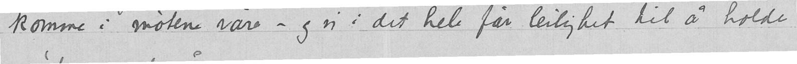komme i møtene våre – og vi i det hele får leilighet til å holde
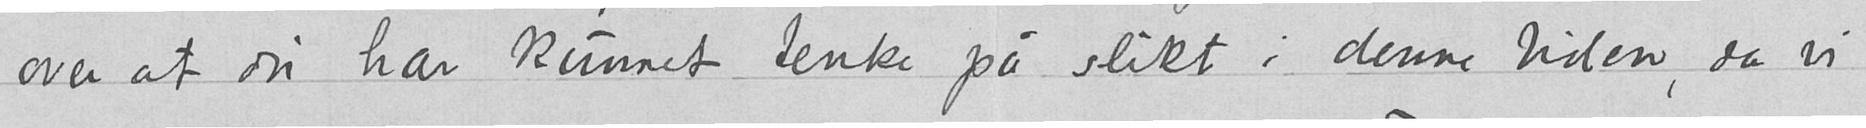over at du har kunnet tenke på slikt i denne tiden, da vi
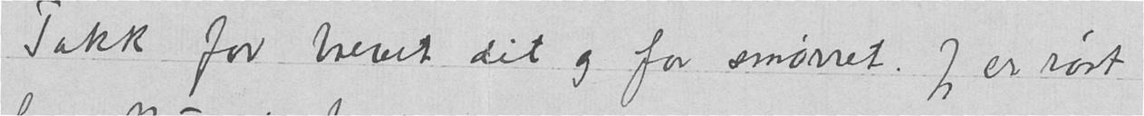Takk for brevet dit og for smørret. Jeg er rørt
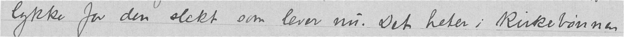lykke for den slekt som lever nu. Det heter i kirkebønnen
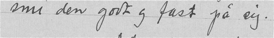smi den godt og fast på sig.
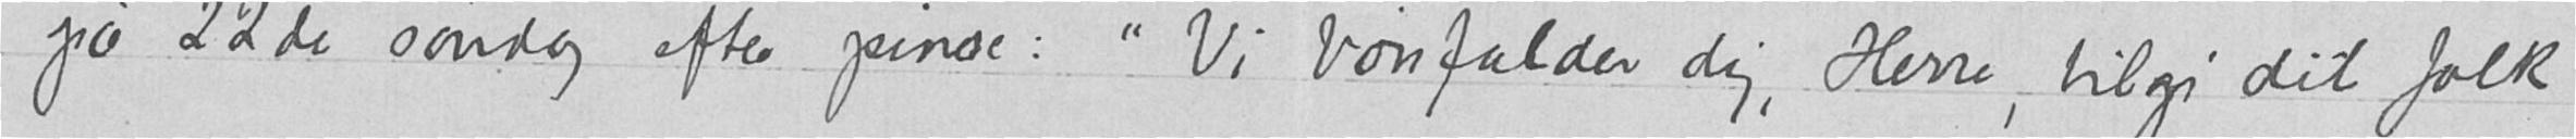på 22de søndag efter pinse: "Vi bønfalder dig, Herre, tilgi dit folk
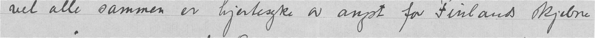vel alle sammen er hjertesyke av angst for Finlands skjebne
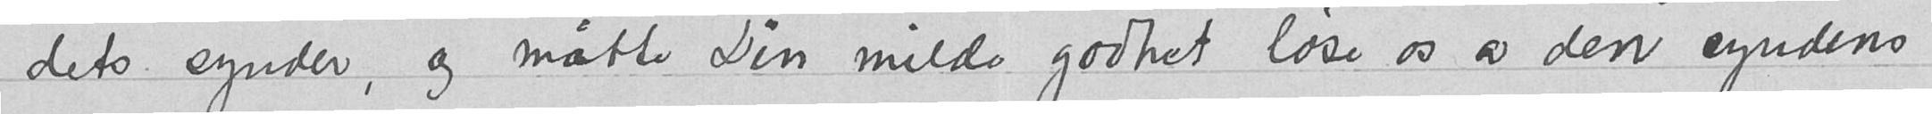dets synder, og måtte Din milde godhet løse os av den syndens
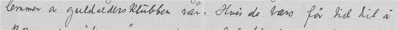lemmer av guldaldersklubben vår. Hvis de bare får tid til å
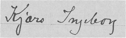Kjære Ingeborg
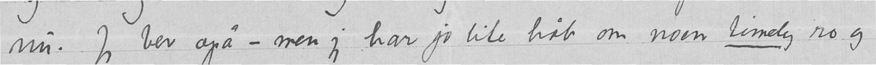nu. Jeg ber også - men jeg har jo lite håb om noen timelig ro og
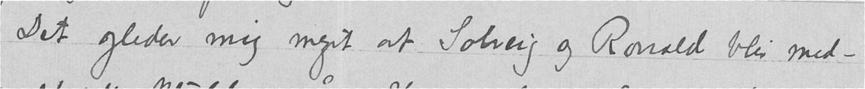Det gleder mig meget at Solveig og Ronald blir med-
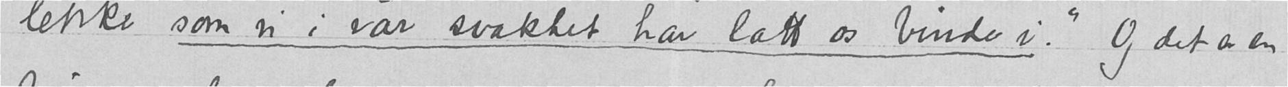lenke som vi i vår svakhet har latt os binde i." Og det er en
